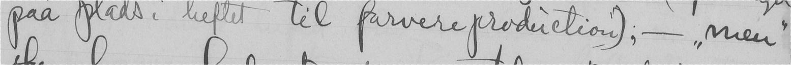paa plads i heftet til farvereproduction); - "men"
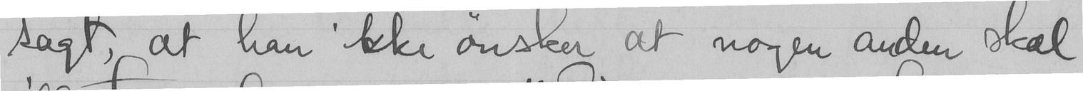sagt, at han ikke ønsker at nogen anden skal
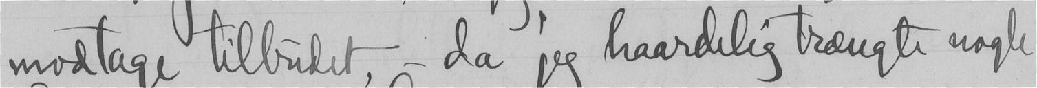modtage tilbudet - da jeg haardelig trængte nogle
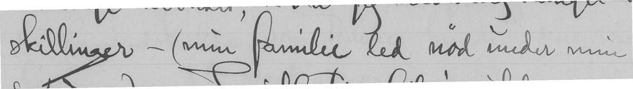skillinger - (min familie led nød under min
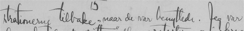strationerne tilbake, naar de var benyttede. Jeg var
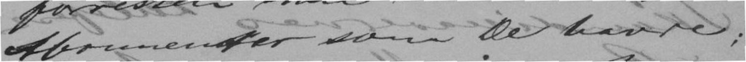Abounenter som De havde;
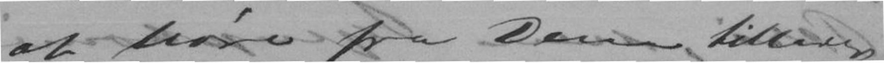at høre fra Dem, tillader
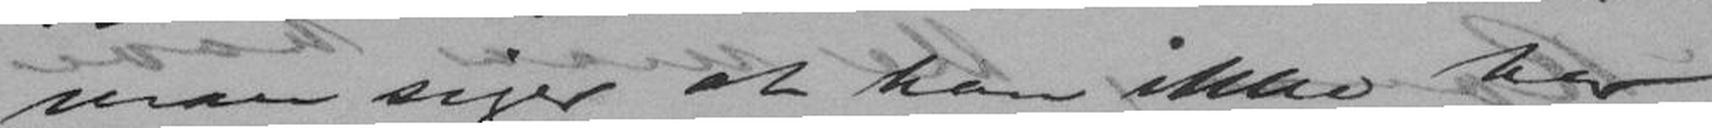man siger at han ikke har
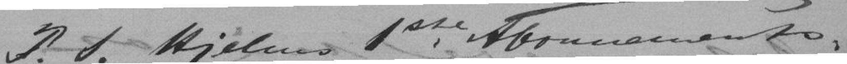P.S. Hjelms 1ste Abounements-
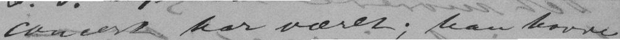concert har været; han havde
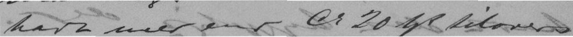havt med end Cr 20 spd tilovers;
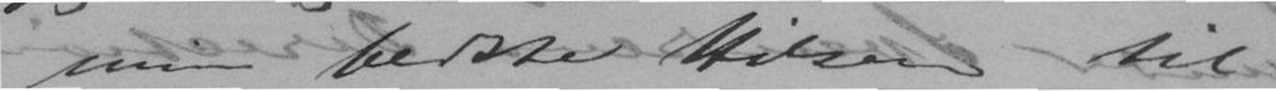min bedste Hilsen til
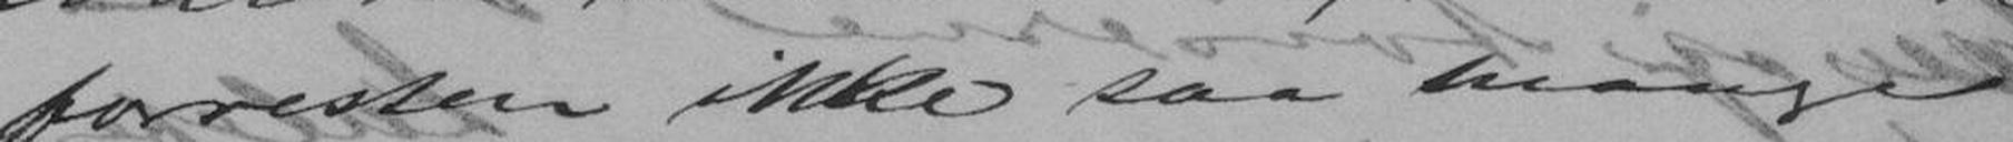forresten ikke saa mange
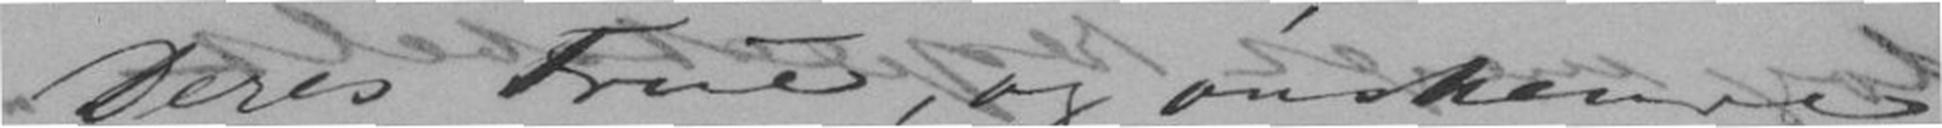Deres Frue, og ønskende
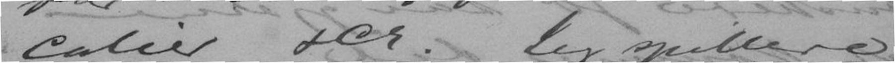calier &cr. Jeg spillede
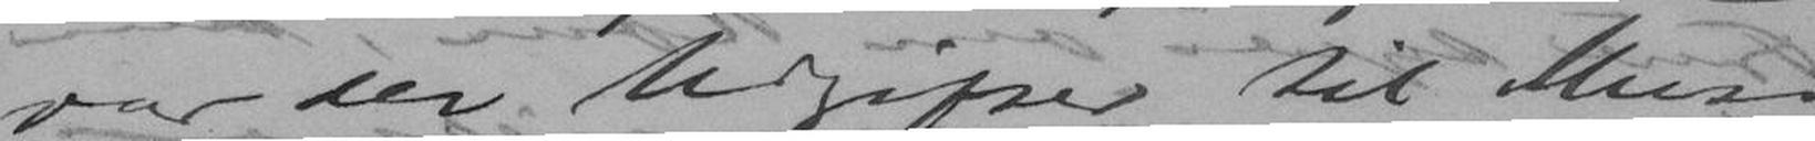var der Udsigter til Musi
